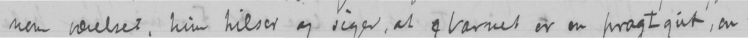nem værelset, hun hilser og siger, at g barnet er en pragtgut, en
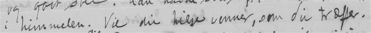i himmelen. Vil du hilse venner, som du træffer.
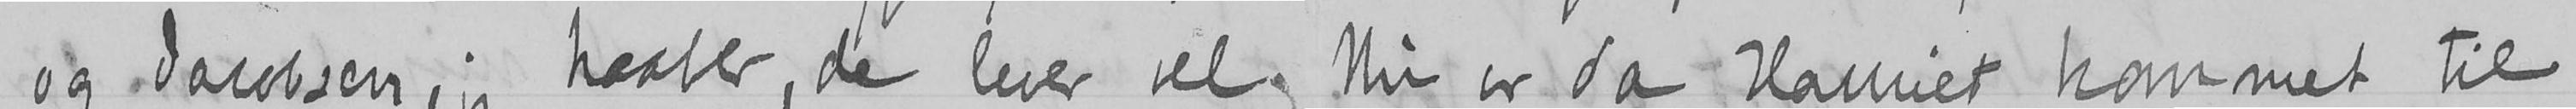og Jacobsen, jeg haaber, de lever vel. Nu er da Harriet kommet til
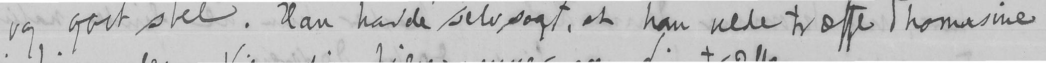og godt stel. Han havde selv sagt, at han vilde træffe Thomasine
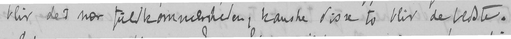blir det nær fuldkommenheden, kanske disse to blir de bedste.
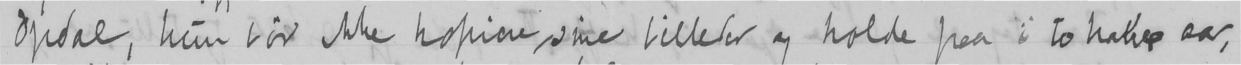Opdal, hun bør ikke kopiere sine billeder og holde paa i to halve aar,
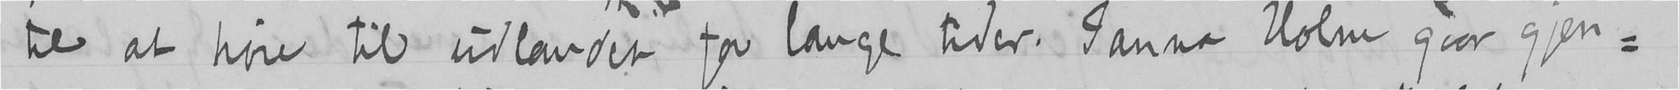til at høre til udlandet for lange tider. Janna Holm gaar gjen-
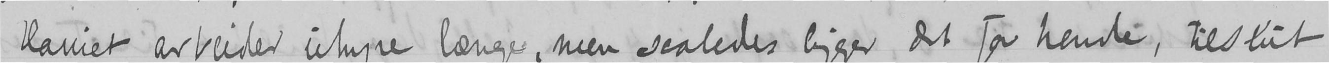Harriet arbeider uhyre længe, men saaledes ligger det for hende, tilslut
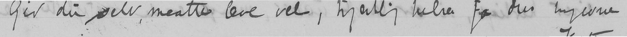Gid du selv maatte leve vel, hjertelig hilsen fra din hengivne
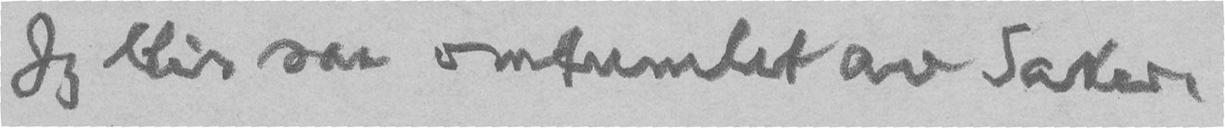Jeg blir saa omtumlet av Saken
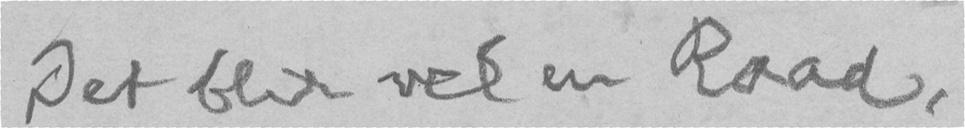Det blir vel en Raad,
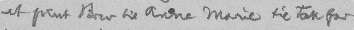et pent Brev til Anne Marie til Tak for
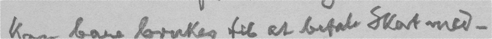kan bare brukes til at betale Skat med.
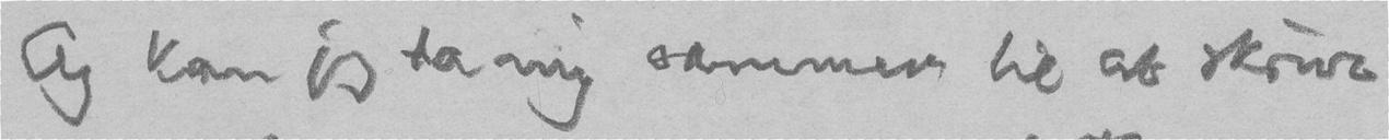Og kan jeg ta mig sammen til at skrive
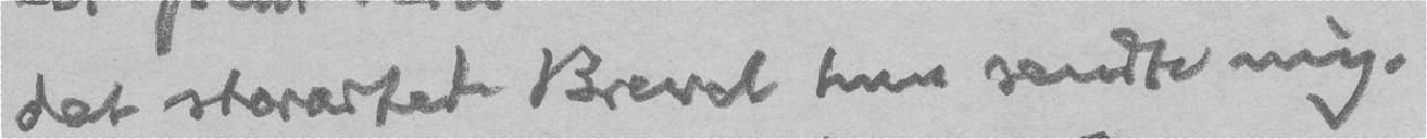det storartet Brevet hun sendte mig.
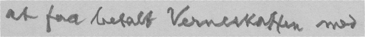at faa betalt Verneskatten med
